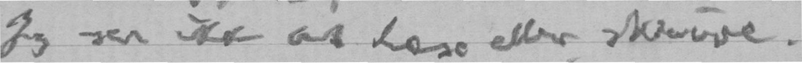Jeg ser ikke at læse eller skrive.
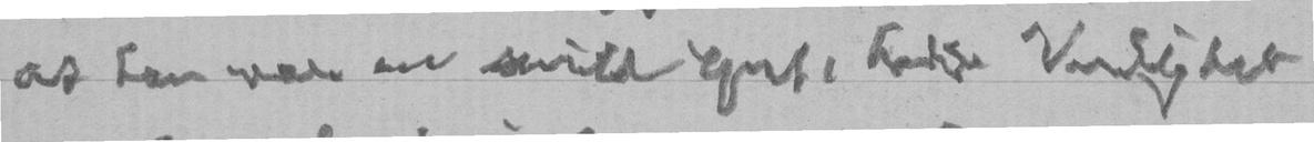at han var en snild Gut, hadde Venlighet
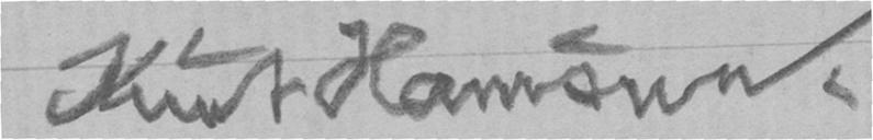Knut Hamsun.
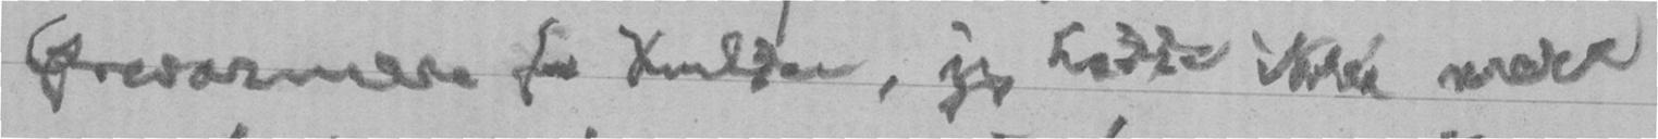Ørevarmere for Kulden, jeg hadde ikke mere
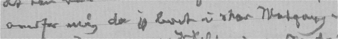overfor mig da jeg levet i stor Motgang.
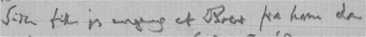Siden fik jeg engang et Brev fra ham da
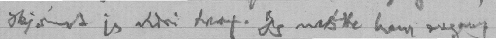skjønt jeg aldri traf. Jeg mødte ham engang
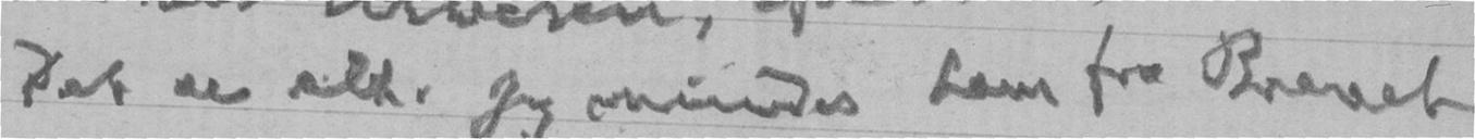Det er alt. Jeg mindes ham fra Brevet
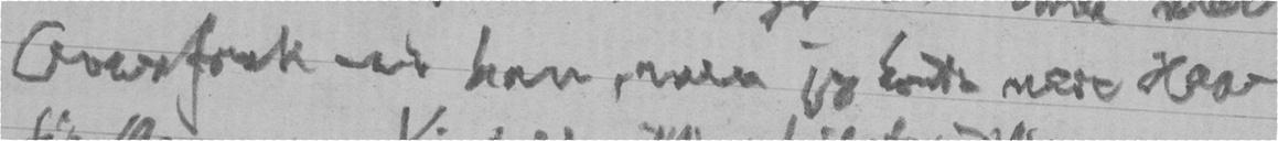Overfrak end han, men jeg hadde mere Haar
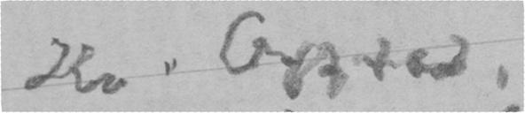Hr. Ofstad,
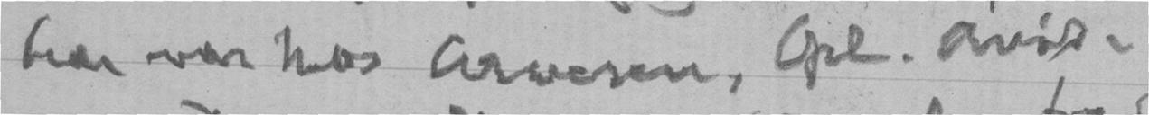han var hos Arvesen, Opl. Avis.
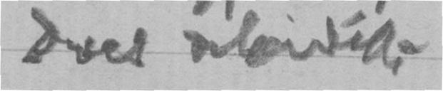Deres ærbødige
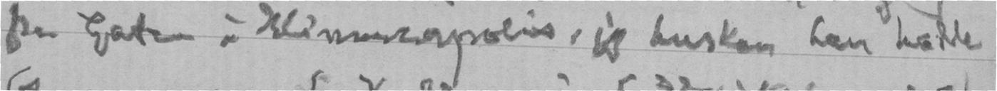paa Gaten i Minneapolis, jeg husker han hadde
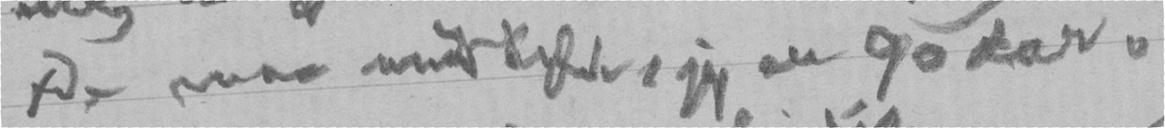De maa undskylde, jeg er 90 Aar.
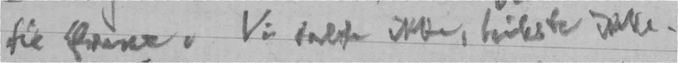til Ørene. Vi talte ikke, hilste ikke.
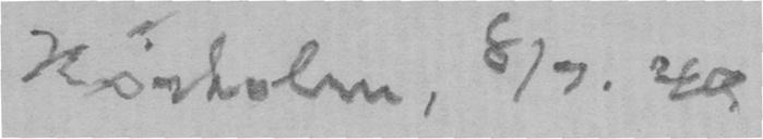Nørholm, 8/9. 49.
